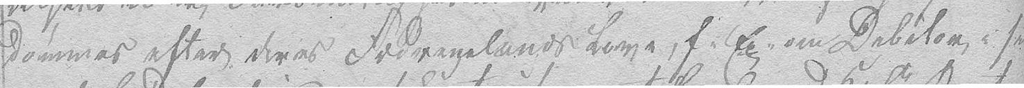dømmes efter deres Fædrenelands Love, f: Ex: om Debitor, i sit
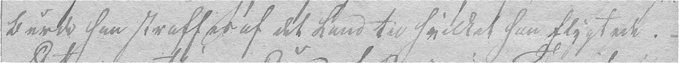burde han straffes af det Land til hvilket han flygtede. –
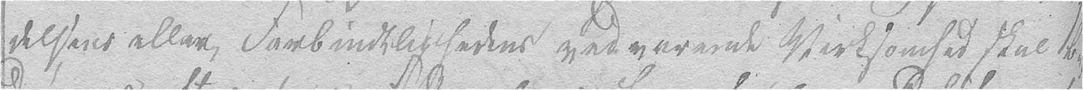delsens eller Forbindtlighedens vedvarende Virksomhed skal be-
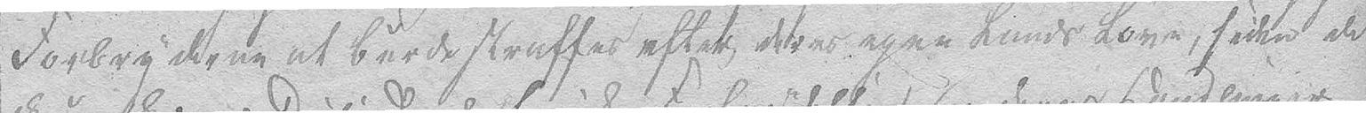Forbryderne at burde straffes efter deres egne Lands Love, siden de
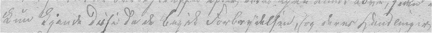kun kjende disse da de begik Forbrydelsen, og deres Handlinger
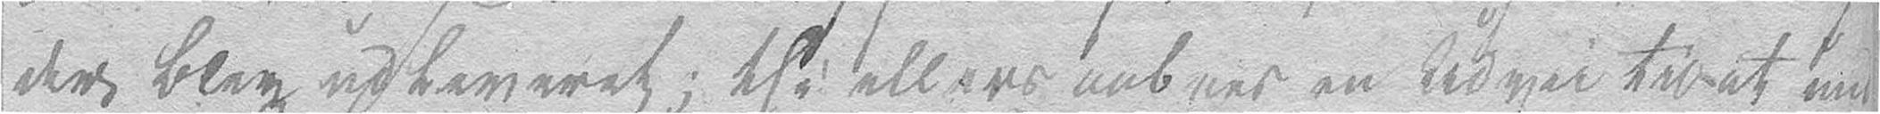der blev udleveret; thi ellers aabnes en Udvei til at und-
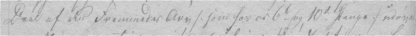Deel af den Fremmedes Arv /:som hos os 6de og 2de Penge:/ udøves
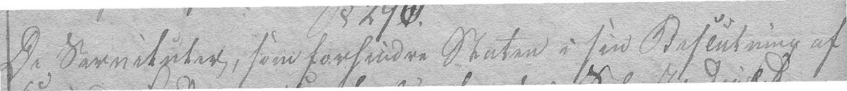De Servituter, som forhindre Staten i sin Beslutning af
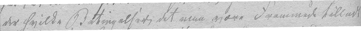der hvilke Betingelser det maa være Fremmede tilladt
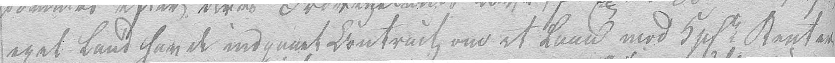eget Land havde indgaaet Contract om et Laan med 5 Pst Renter
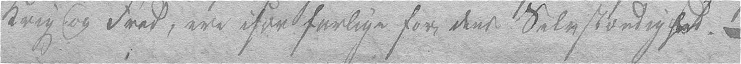Krig og Fred, ere især farlige for dens Selvstændighed.–
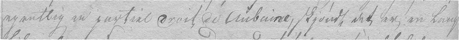egentlig en partiel droit de Auburne, skjøndt det er en langt
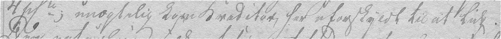4 Pst; unægtelig kom Creditor her uforskyldt til at lide.
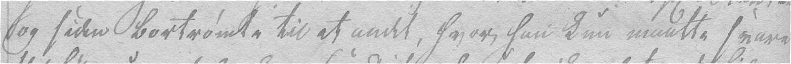og siden bortrømte til et andet, hvor han kun maatte svare
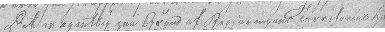Det er egentlig paa Grund af Regjeringen Territorial-Høi-
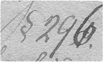§ 296.
